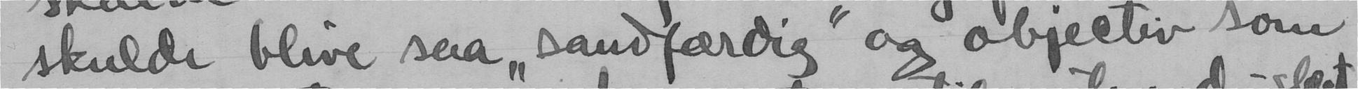skulde blive saa "sandfærdig" og objectiv som
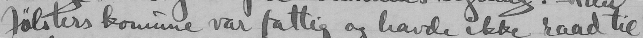Jølsters komme var fattig og havde ikke raad til
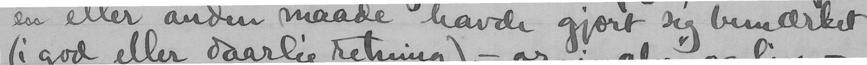en eller anden maade havde gjort sig bemærket
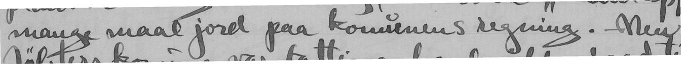mange maal jord paa komunens regning. - Men
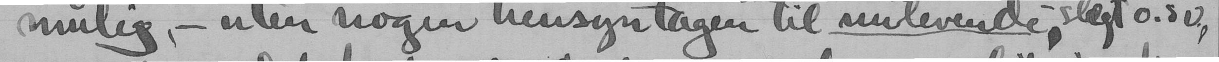mulig, - uten nogen hensyntagen til nulevende, slegt o.s.v.,
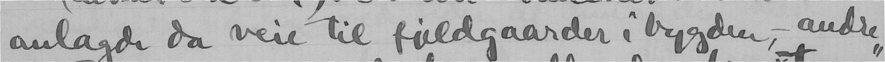anlagde da veie til fjeldgaarder i bygden, - andre
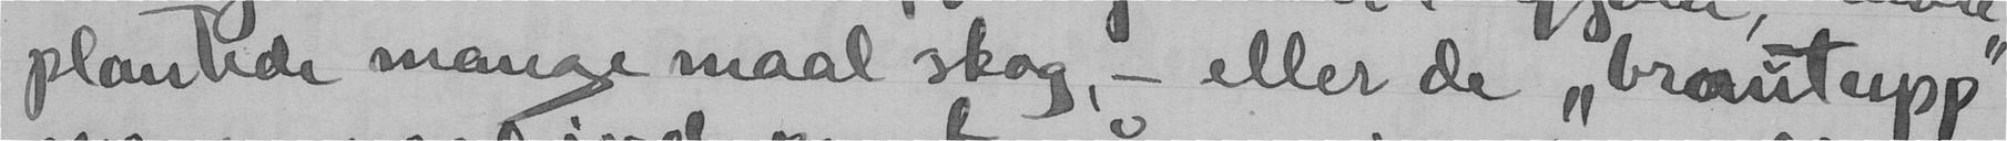plantede mange maal skog, - eller de "braut upp"
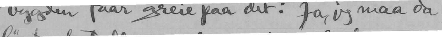bygden faar greie paa det: Ja, jeg maa da
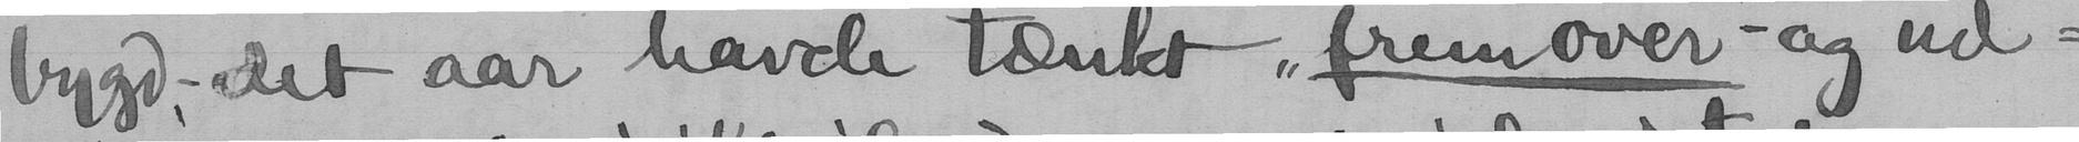bygd,- det aar havde tænkt "fremover" - og ud-
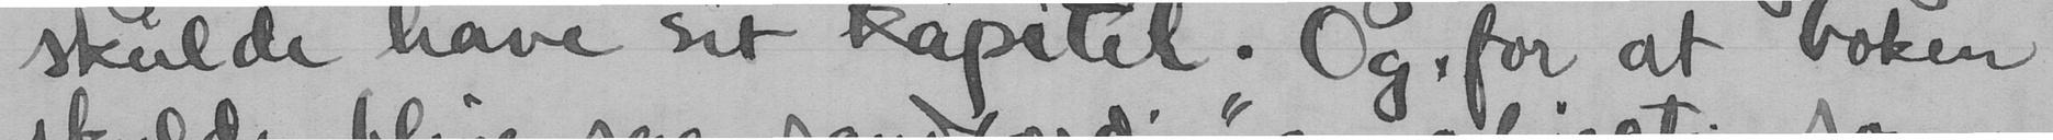skulde have sit kapitel. Og, for at boken
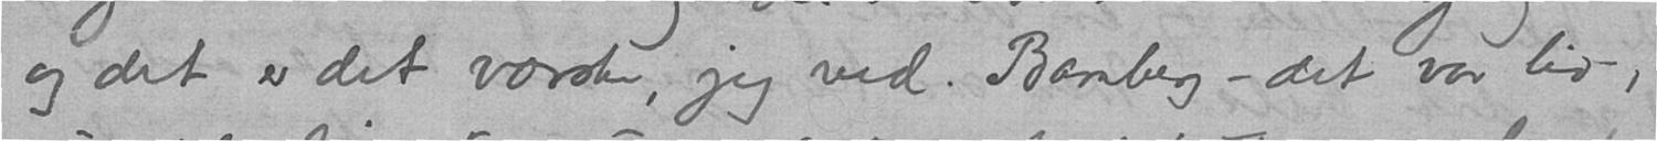og det er det værste, jeg ved. Bamberg - det var liv,
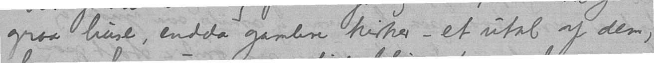graa huse, endda gamlere kirker - et utal af dem,
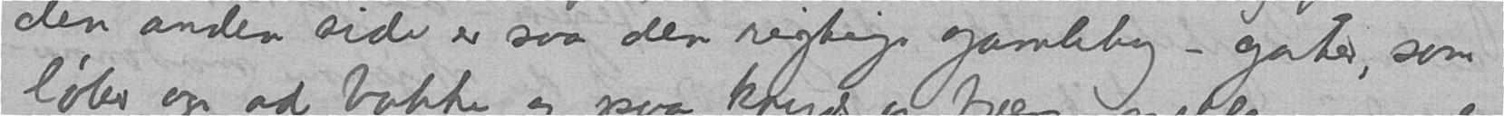den anden side er saa den rigtige gamleby - gater, som
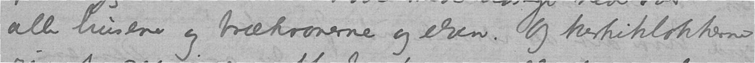alle husene og trækronerne og elven. Og kirkeklokkerne
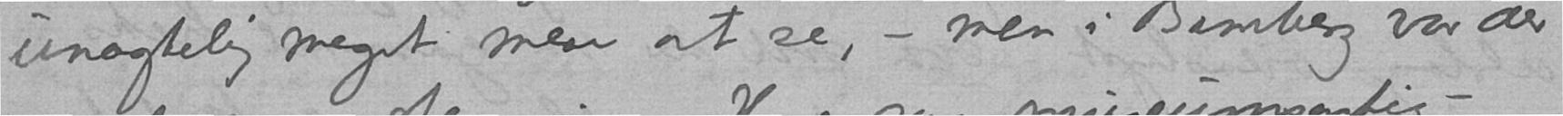unægtelig meget mere at se, - men i Bamberg var der
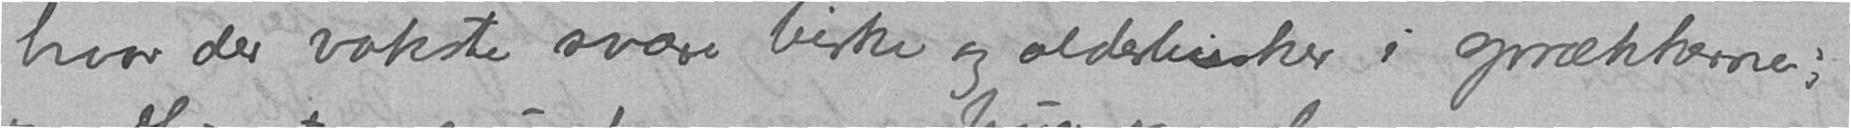hvor der vokste svære birke og olderbusker i sprækkerne;
